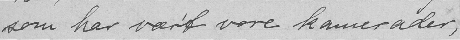som har vært vore kamerader,
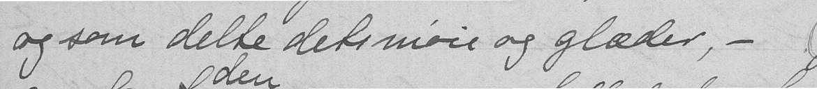og som delte dets møie og glæder, -
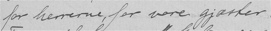for herrerne, for vore gjæster!
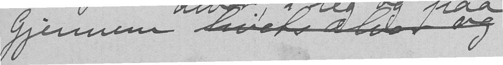gjennem livets alvor og
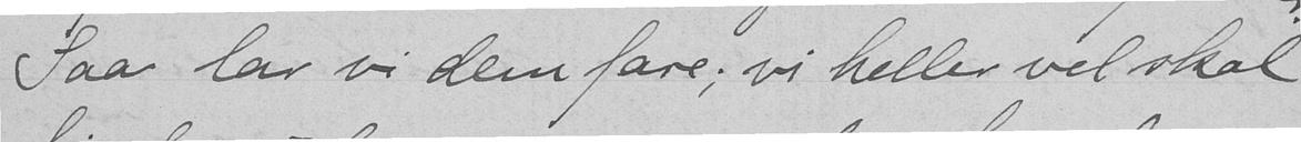Saa lar vi dem fare; vi heller vel skal
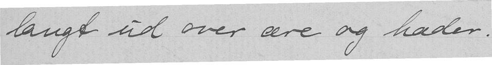langt ud over ære og hæder.
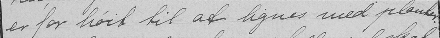er for høit til at lignes med planter.
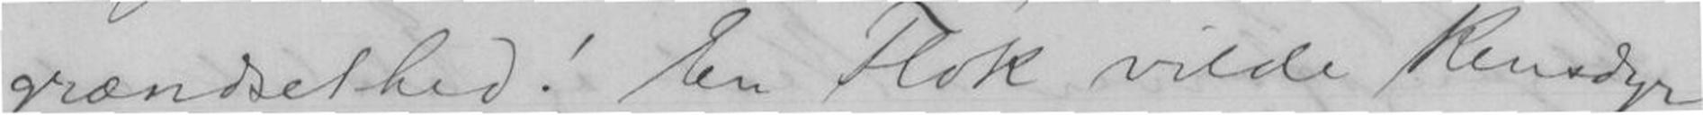grændsethed! En Flok vilde Rensdyr
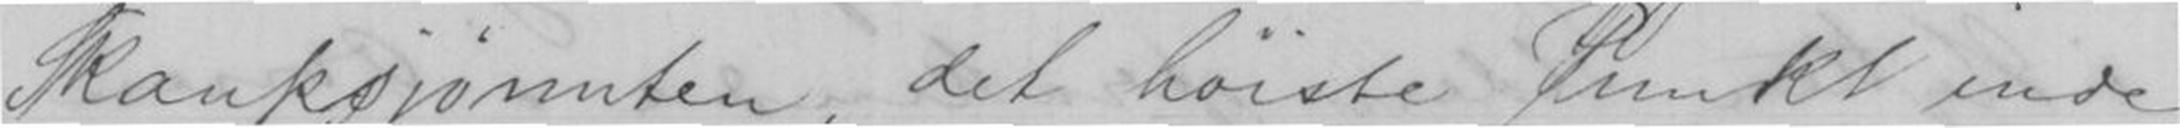Skaupsjønuten, det høiste Punkt inde
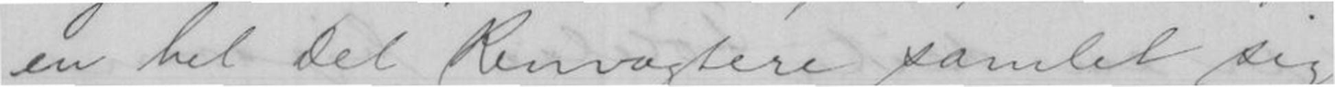en hel Del Renvogtere samlet sig
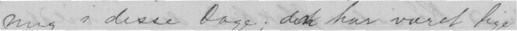mig i disse Dage; den har været lige
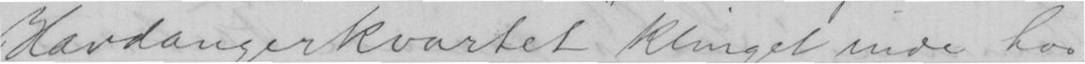Hardangerkvartet klinget inde hos
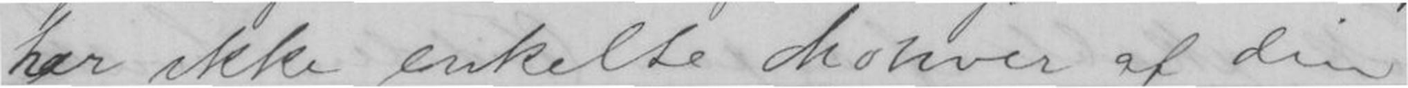har ikke enkelte Motiver af din
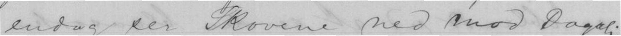endog ser Skovene ned mod Dagali
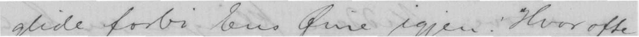glide forbi Ens Øine igjen! Hvor ofte
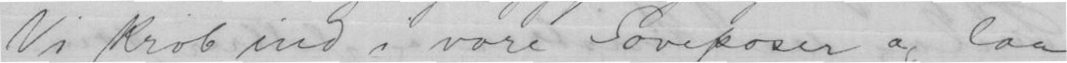Vi Krøb ind i vore Soveposer og laa
